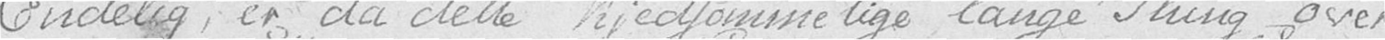Endelig, er da dette kjedsommelige lange Thing over,
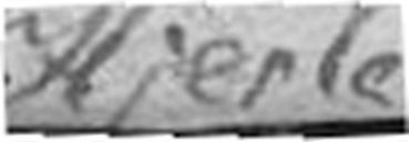Hjerte.
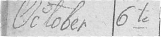October 6te
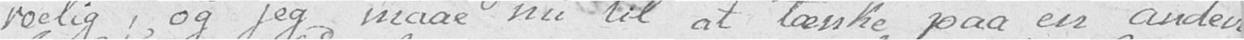roelig, og jeg maae nu til at tænke paa en anden
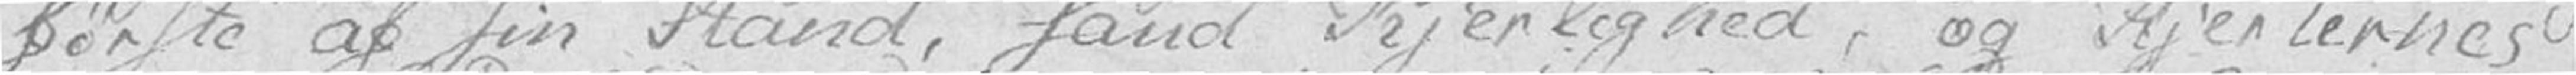første af sin Stand, sand Kjerlighed, og Hjerternes
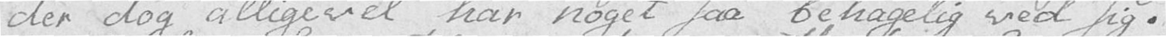der dog alligevel har noget saa behagelig ved sig.
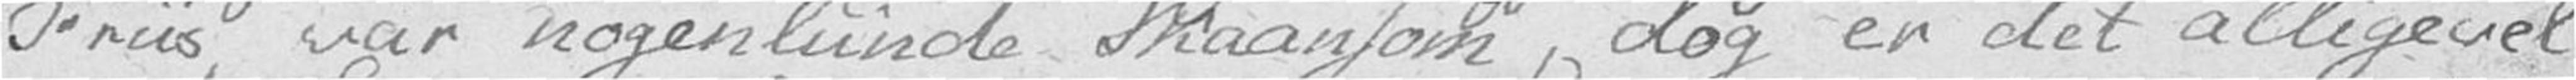Friis var nogenlunde Skaansom, dog er det alligevel
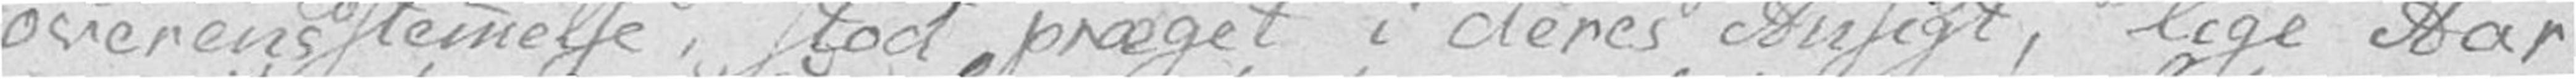overensstemmelse, stod præget i deres Ansigt, lige Aar
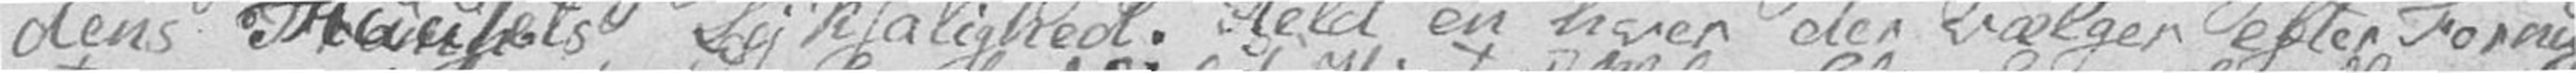dens Huusets Lyksalighed. Held en hver der vælger efter fornuf
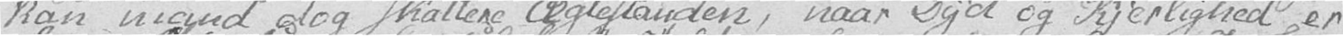kan mand dog skattene Ægtestanden, naar Dyd og Kjerlighed er
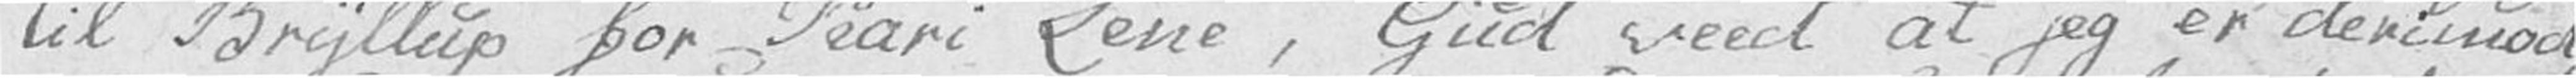til Bryllup for Kari Lene, Gud veed at jeg er derimod,
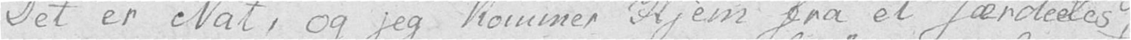Det er Nat, og jeg kommer Hjem fra et særdeeles
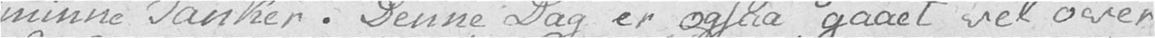minne Tanker. Denne Dag er ogsaa gaaet vel over,
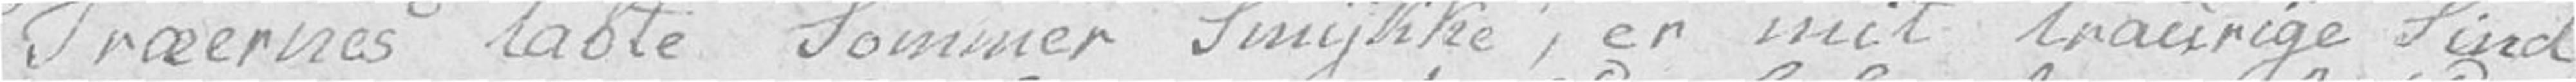Træernes tabte Sommer Smykke, er mit traurige Sind,
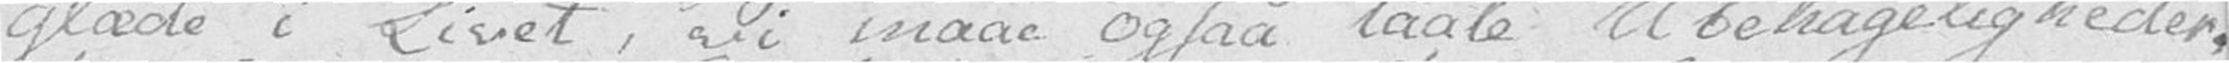glæde i Livet, vi maae ogsaa taale Ubehageligheder,
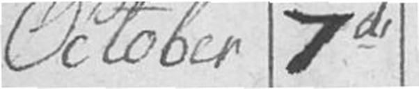October 7de
